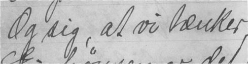Og sig, at vi tænker
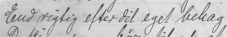End rigtig efter dit eget behag
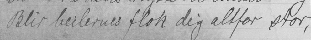Blir beilernes flok dig altfor stor,
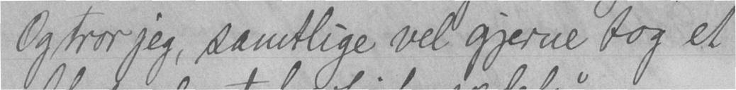Og tror jeg, samtlige vel gjerne tog et
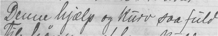Denne hjælp og kurv saa fuld
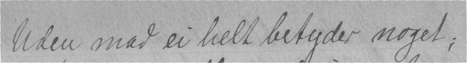Uden mad ei helt betyder noget;
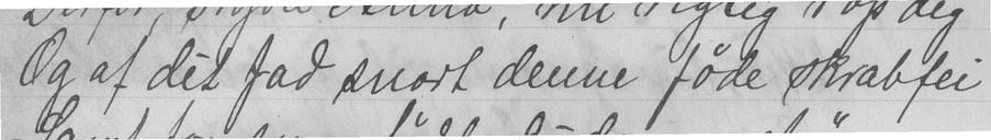Og af det fad snart denne føde skrabfei
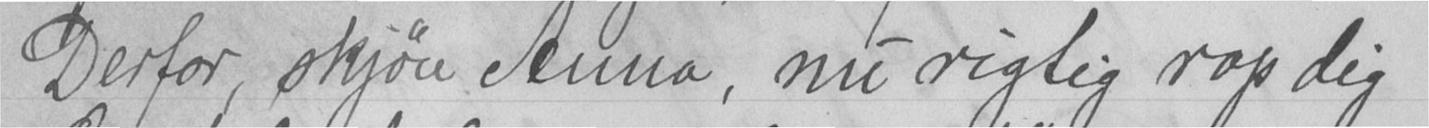Derfor, skjøn Anna, nu rigtig rop dig
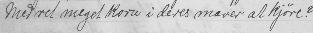Med ret meget korn i deres maver at kjøre?
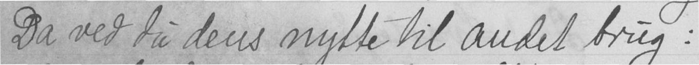Da ved du dens nytte til andet brug:
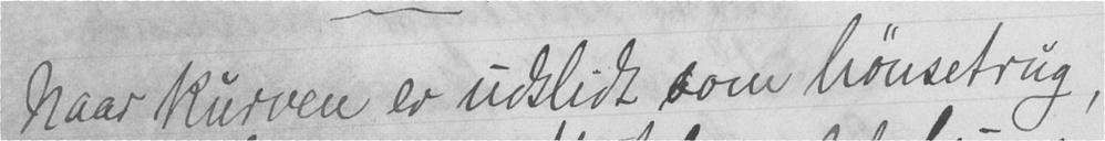Naar kurven er udslidt som hønsetrug,
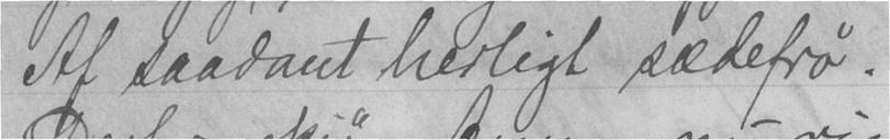Af saadant herligt sædefrø.
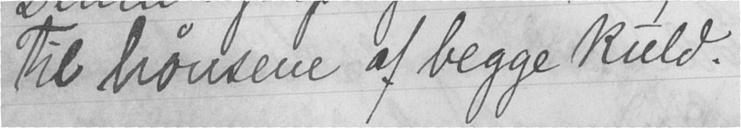til hønsene af begge kuld.
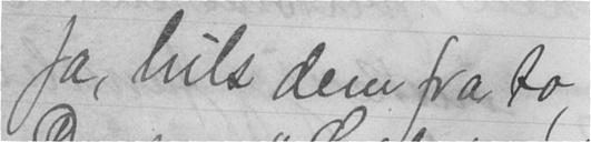Ja, hils dem fra to,
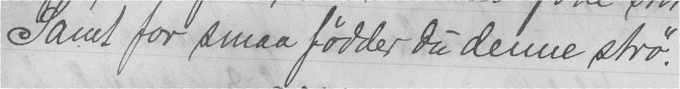Samt for smaa fødder du denne strø.
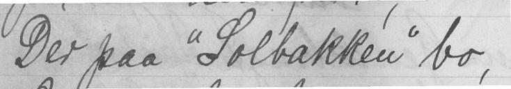Der paa "Solbakken" bo,
